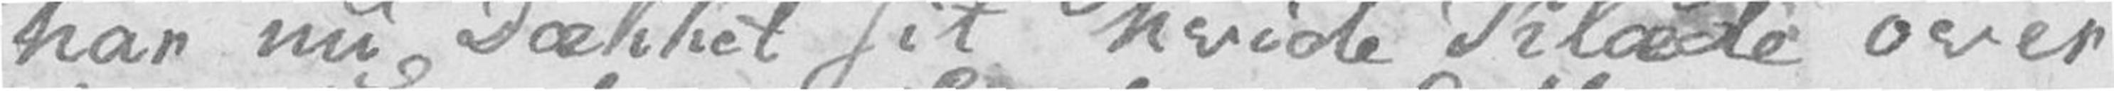har nu Dækket sit hvide Klæde over
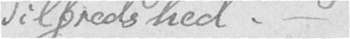Tilfredshed. –
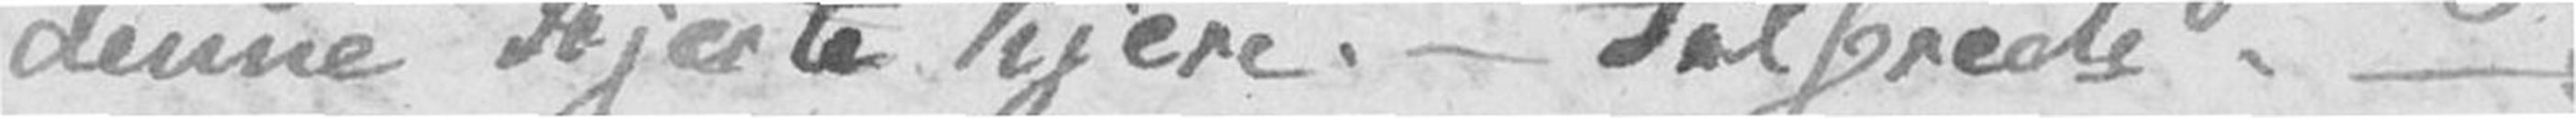denne Hjerte kjere. – Tilfreds. –
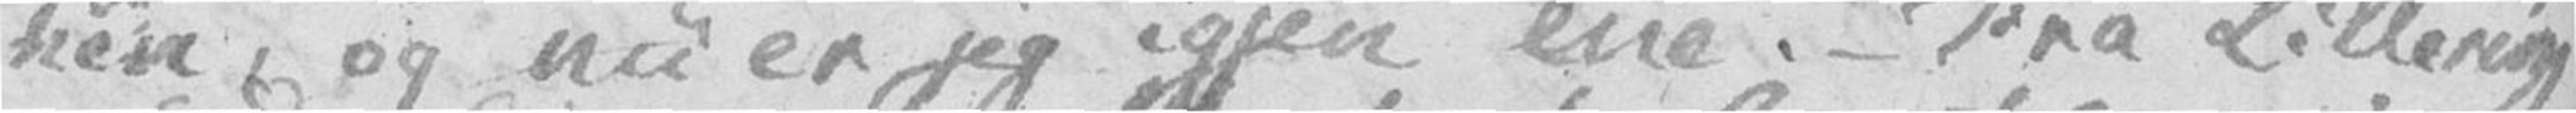hen, og nu er jeg igjen ene. – Fra Lillering
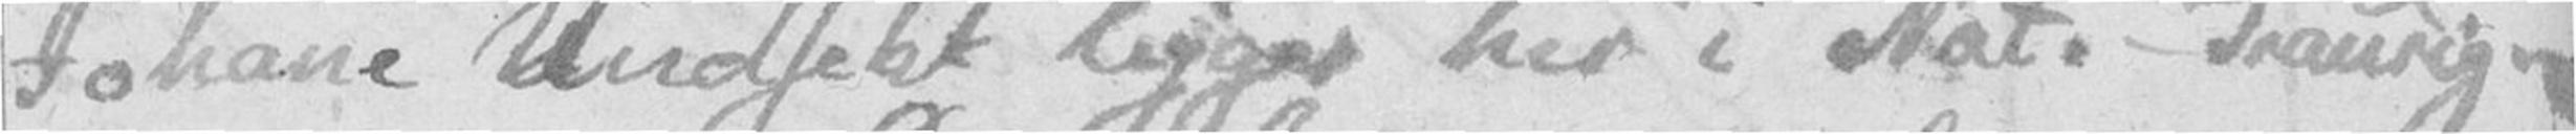Johane Undfelt ligger her i Nat. – Traurig.
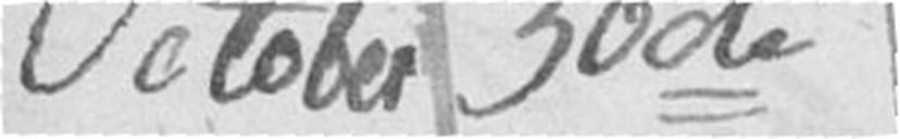October 30de
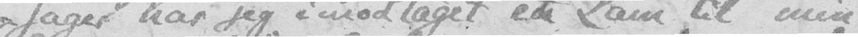-sager har jeg imodtaget et Lam til min
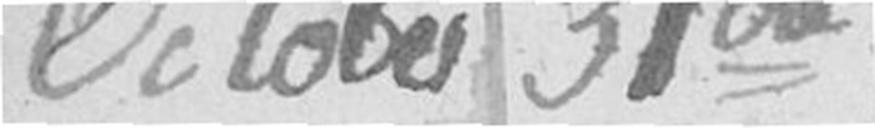October 31de
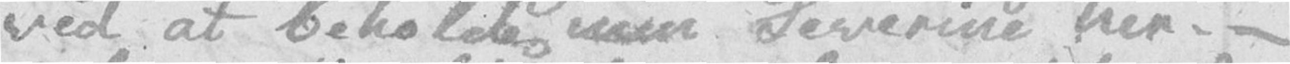ved at beholde min Severine her. –
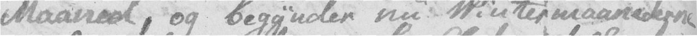Maaned, og begynder nu Vintermaanederne
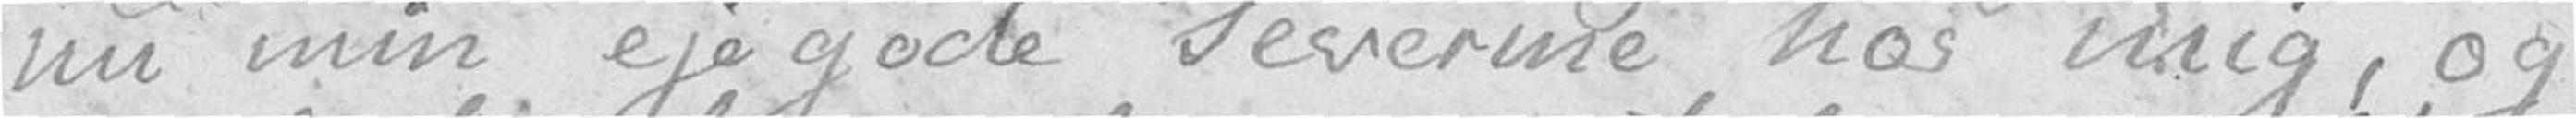nu min eje gode Severine hos mig, og
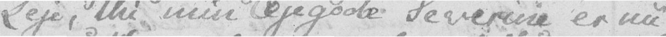Leje, thi min ejegode Severine er nu
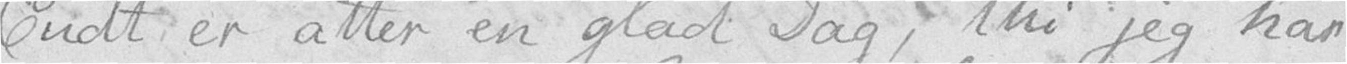Endt er atter en glad Dag, thi jeg har
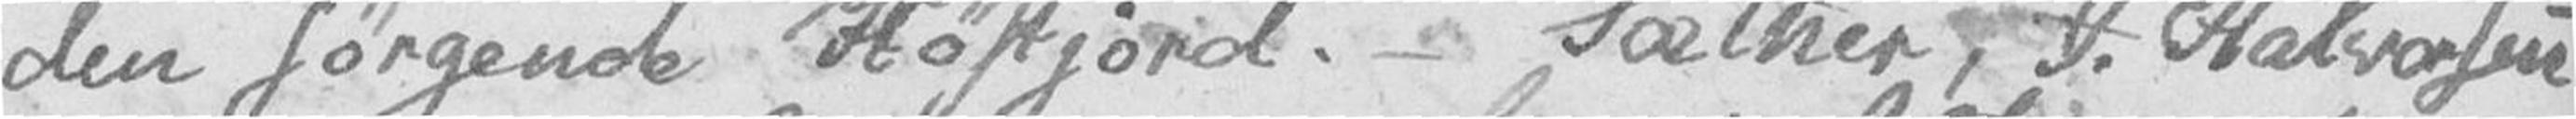den sørgende Høstjord. – Sæther, J. Halvorsen
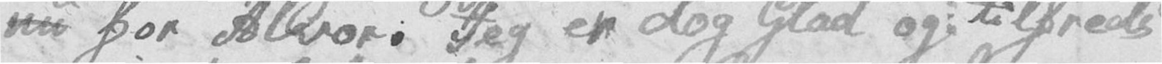nu for Alvor. Jeg er dog Glad og tilfreds
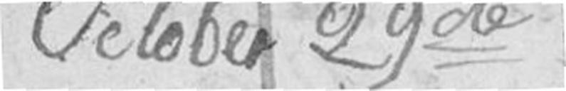October 29de
